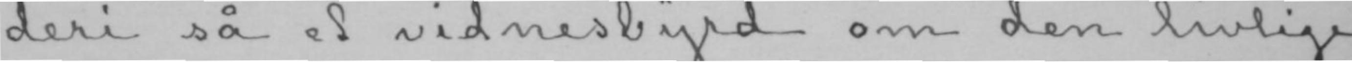deri så et vidnesbyrd om den livlige
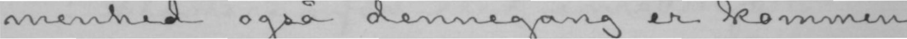menhed også dennegang er kommen
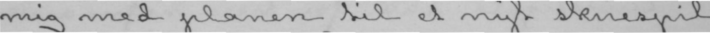mig med planen til et nyt skuespil
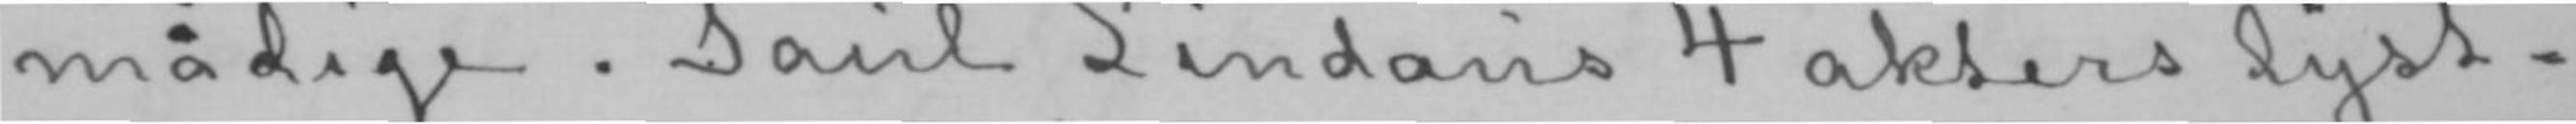mådige. Paul Lindaus 4 akters lyst-
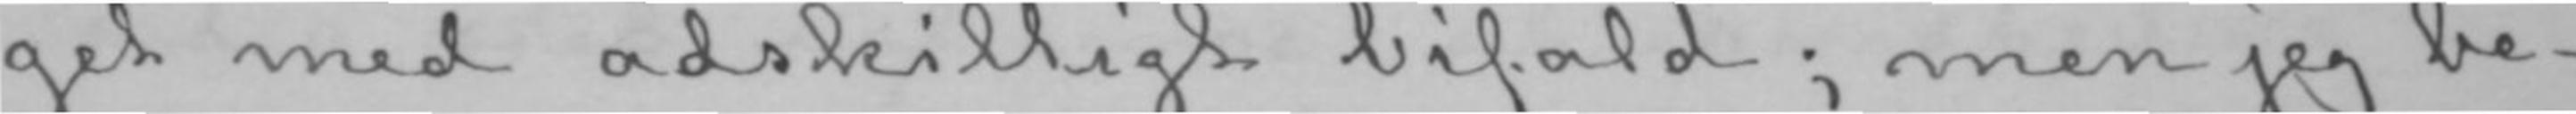get med adskilligt bifald; men jeg be-
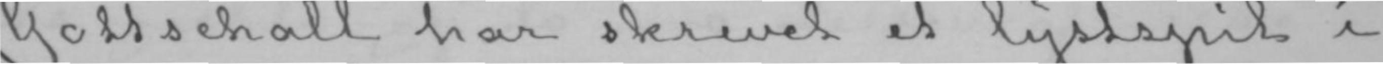Gottschall har skrevet et lystspil i
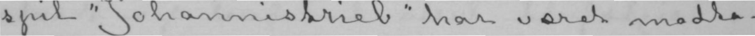spil «Johannistrieb» har været modta-
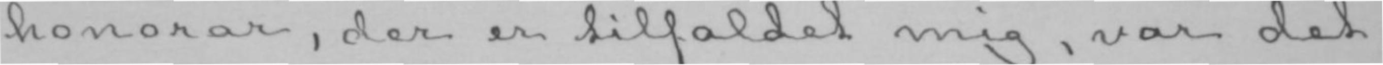honorar, der er tilfaldet mig, var det
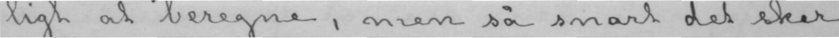ligt at beregne, men så snart det sker
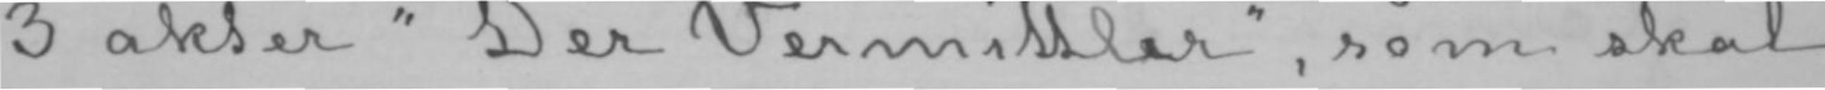3 akter «Der Vermittler», som skal
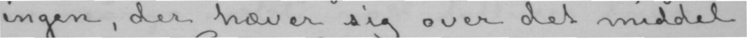ingen, der hæver sig over det middel-
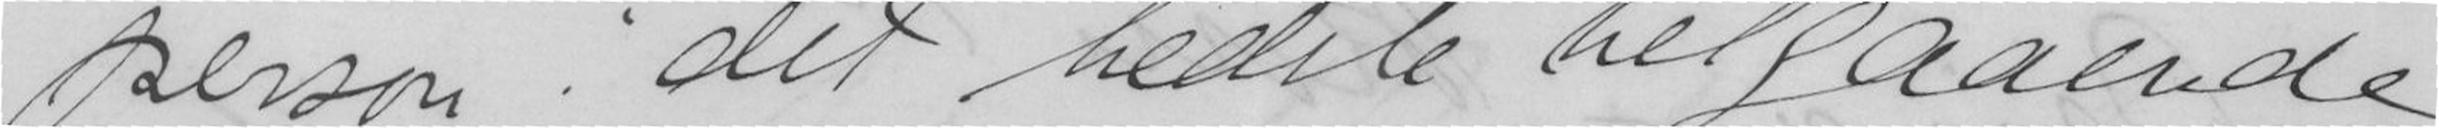person i det bedste velgaaende,
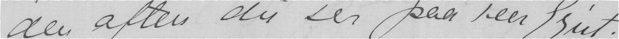den aften du ser paa Peer Gynt.
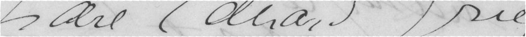Kjære Edvard Grieg
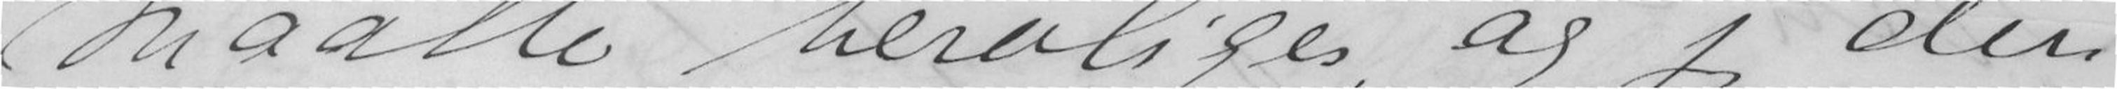maatte beroliges, og jeg den
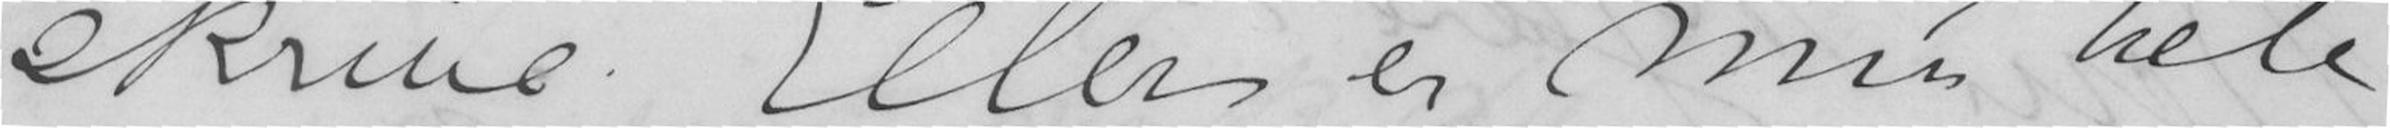skrive. Ellers er min hele
